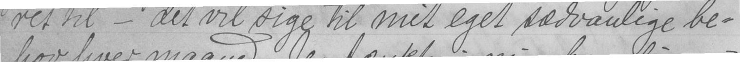ret til - det vil sige til mit eget sædvanlige be-
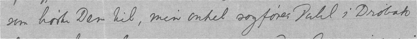som hørte Dem til, min onkel sagfører Dahl i Drøbak
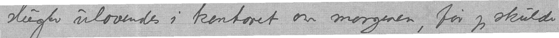slugte ulovendes i kontoret om morgenen, før jeg skulde
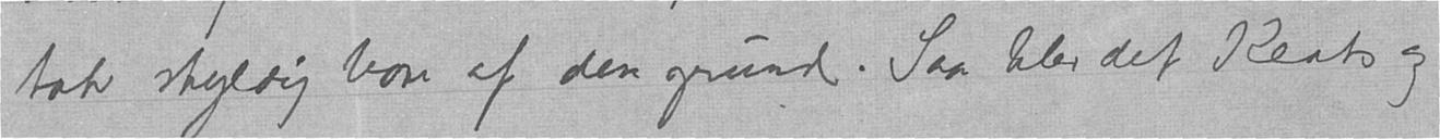tak skyldig bare af den grund. Saa blev det Keats og
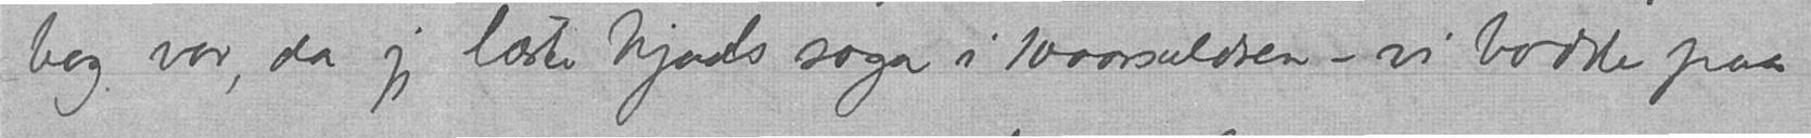bog var, da jeg læste Njaals saga i 10aarsaldren - vi bodde paa
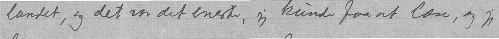landet, og det var det eneste, jeg kunde faa at læse, og jeg
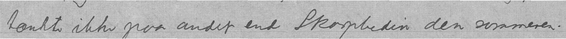tænkte ikke paa andet end Skarphedin den sommeren.
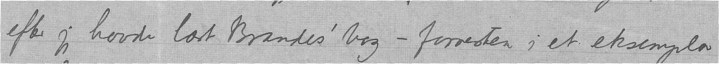- efter jeg havde læst Brandes' bog – forresten i et eksemplar
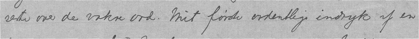serte over de vakre ord. Mit første ordentlige indryk af en
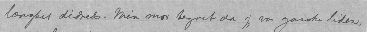længtes didned-. Min mor tegnet da jeg var ganske liden,
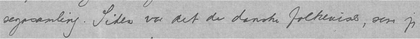sagasamling. Siden var det de danske folkeviser, som jeg
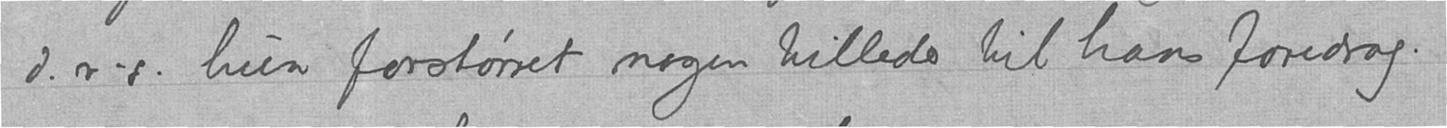d.v.s. hun forstørret nogen billeder til hans foredrag.
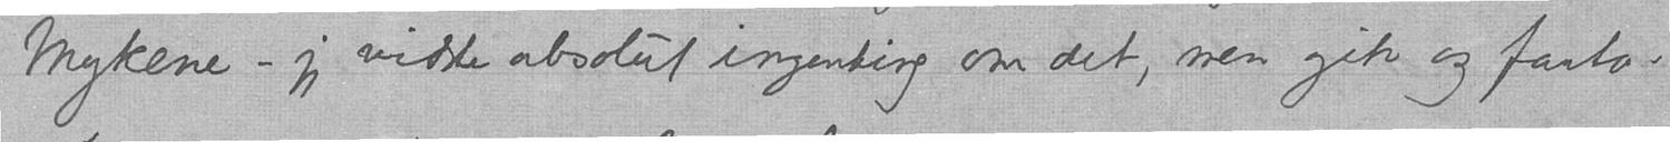Mykene - jeg vidste absolut ingenting om det, men gik og fanta-
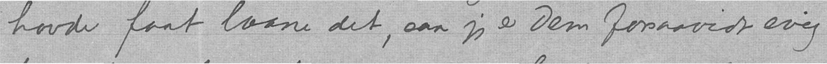havde faat laane det, saa jeg er Dem forsaavidt evig
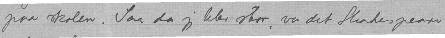paa skolen. Saa da jeg blev stor, var det Shakespeare
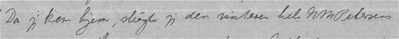Da jeg kom hjem, slugte jeg den vinteren hele N M Petersens
